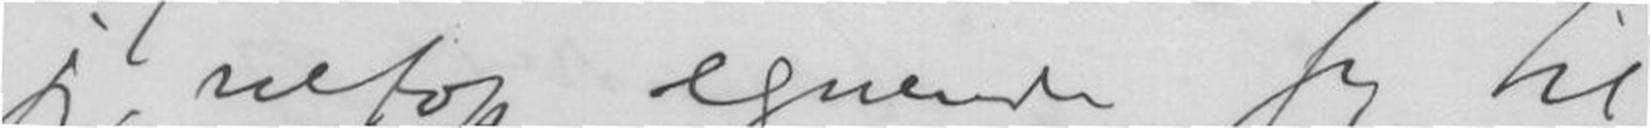jeg netop egnede sig til
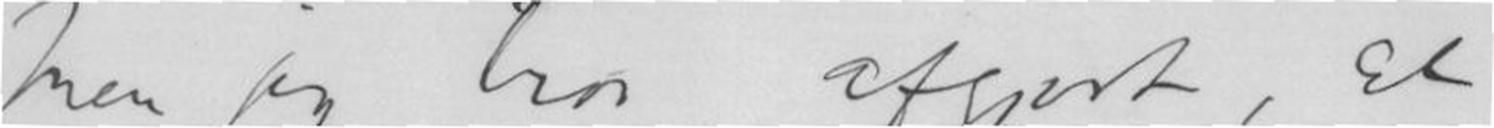men jeg tror afgjort, at
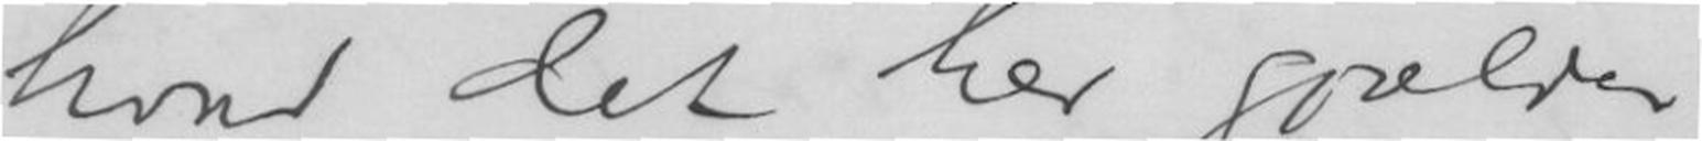hvad det her gjælder
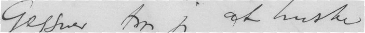Gessne tror jeg at huske
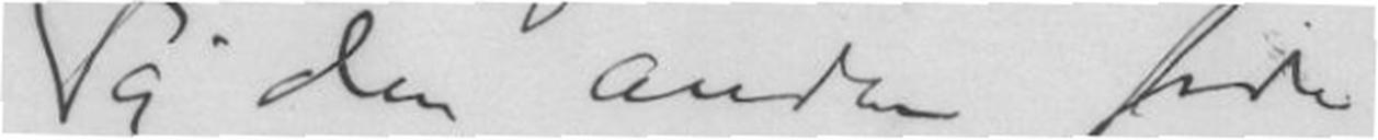På den anden side
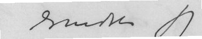sendte jeg
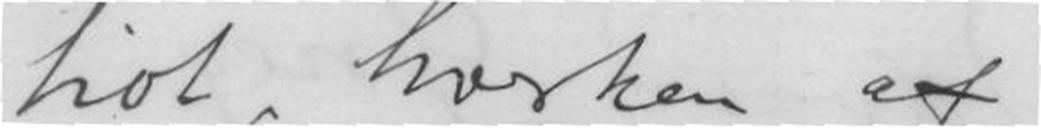liot, hvorhen af
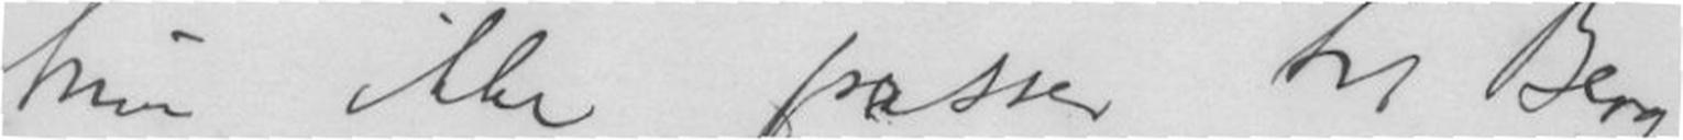hun ikke passer til Berg-
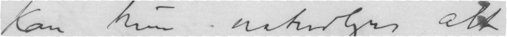kan hun naturligvis alt
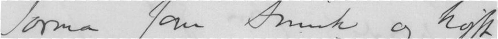Sorna Som Smuk og højt
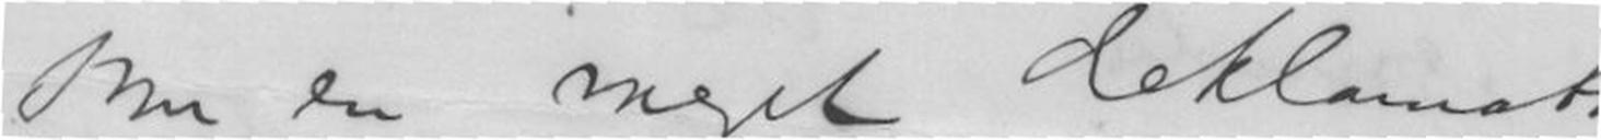hun er meget deklamat
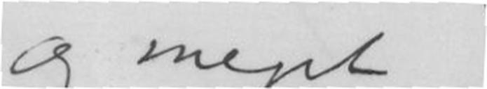og meget
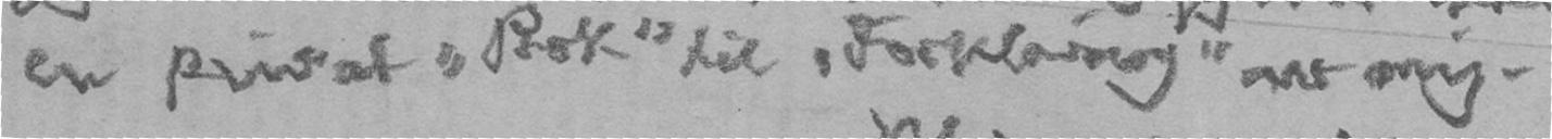en privat "Bok" til "Forklaring" av mig.
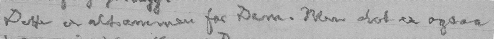Dette er altsammen for Dem. Men det er ogsaa
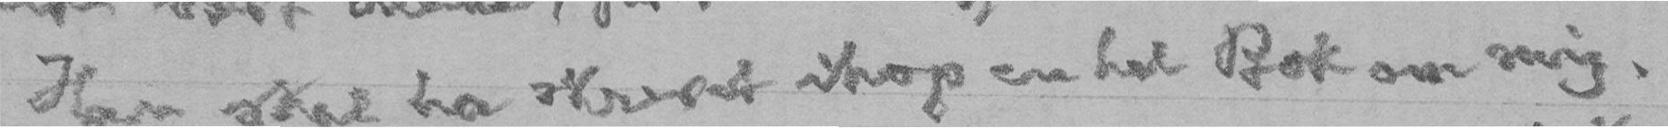Han skal ha skrevet ihop en hel Bok om mig.
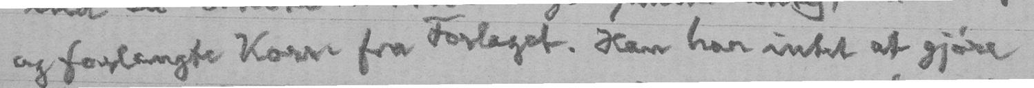og forlangte Korr fra Forlaget. Han har intet at gjøre
